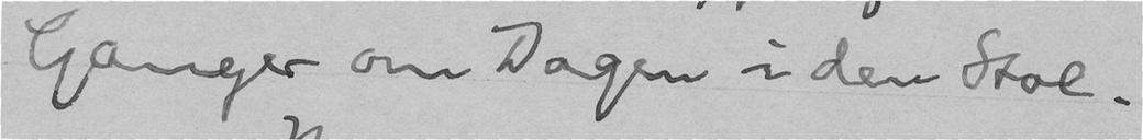Ganger om Dagen i den Stol.
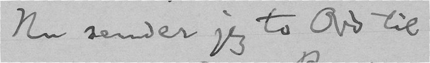Nu sender jeg to Ord til
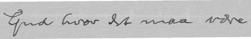Gud hvor det maa være
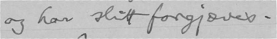og har slitt forgjæves.
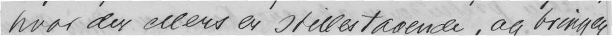hvor der ellers er stillestaaende, og bringer
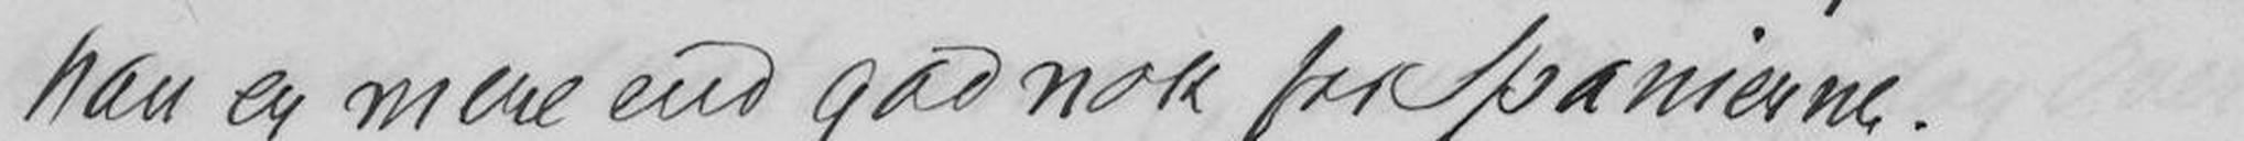han er mere end god nok for Spanierne.
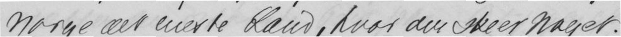Norge det eneste Kand, hvor der skeer noget.
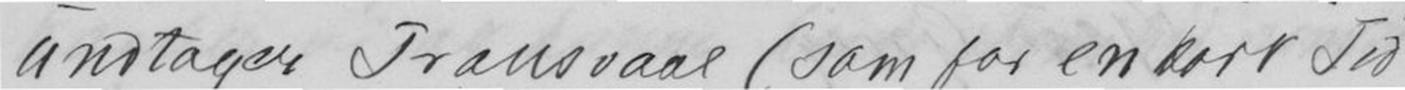undtager vaal (som for en kort Tid
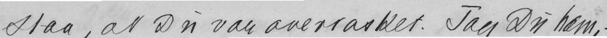Staa, at Du var overrasket. Tag Du hæm,-
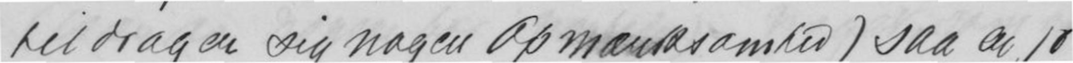tildrager sig nogen Opmærksomhed) saa er jo
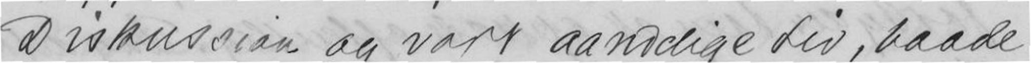Diskussion og vort aandige Liv, baade
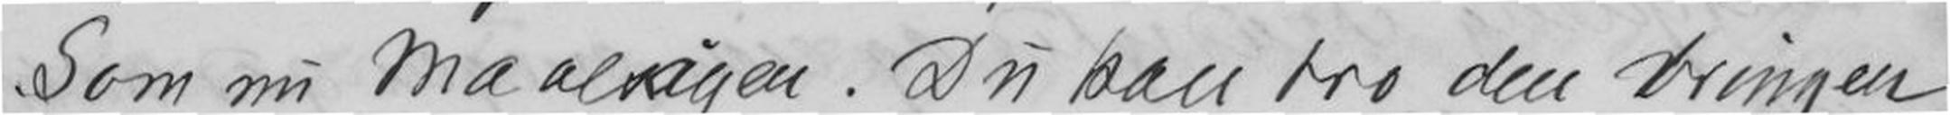Som nu Maalrigen. Du kan tro den bringer
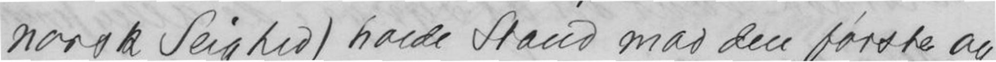norsk Seighed) holde Strand mod den første og
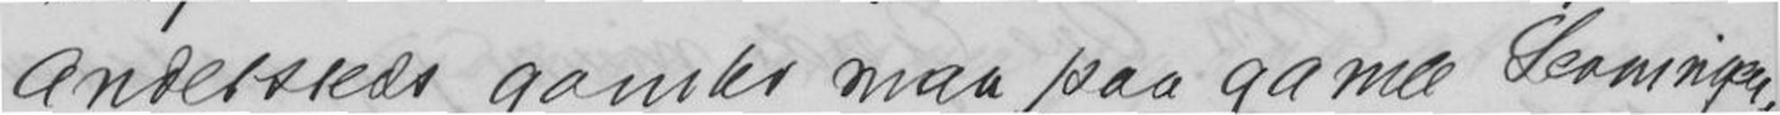andetsteds maa paa gamle
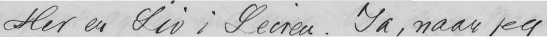Her er Liv i Leiren. Ja, naar jeg
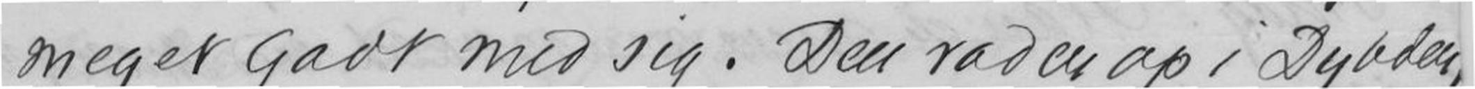meget godt med sig. Den roder op i Dybden,
